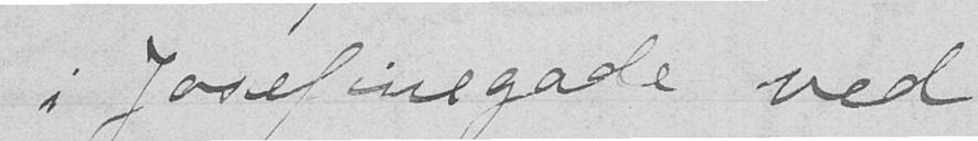i Josefinegade ved
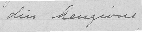din hengivne
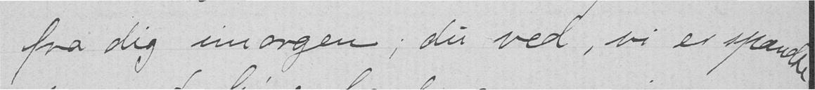fra dig imorgen; du ved, vi er spænde
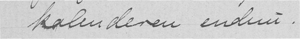kalenderen endnu.
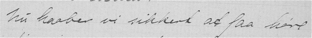Nu haaber vi sikkert at faa høre
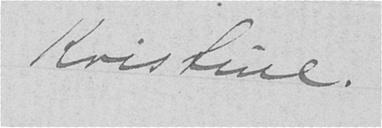Kristine.
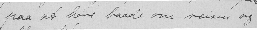paa at høre baade om reisen og
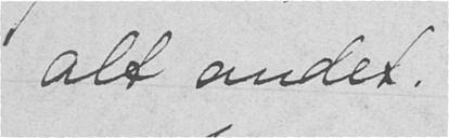alt andet.
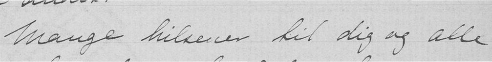Mange hilsener til dig og alle
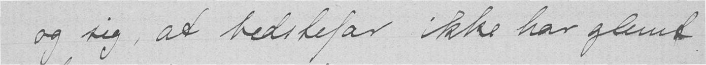og sig, at bedstefar ikke har glemt
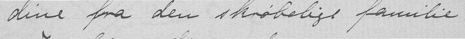dine fra den skrøbelige familie
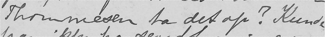Thommesen ta det op? Kunde
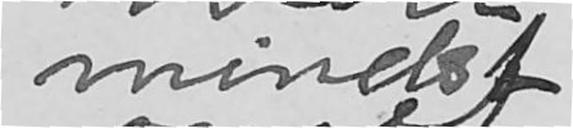mindst
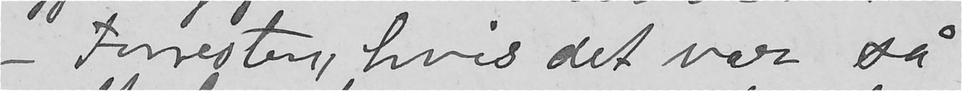- Forresten, hvis det var så
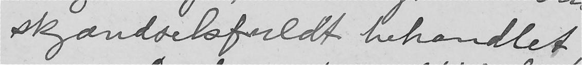skjændselsfuldt behændlet,
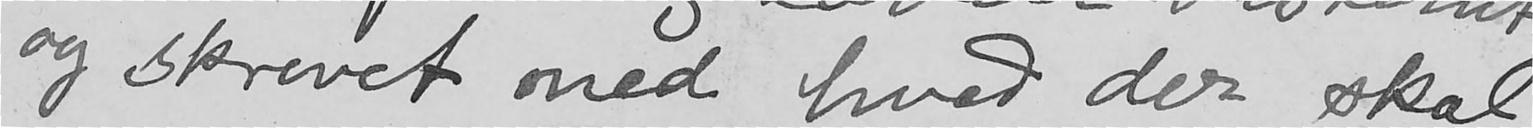og skrevet ned hvad der skal
